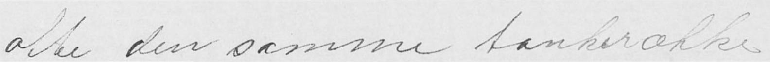ofte den same tankerække
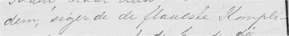dem, siger de de flaueste Kompli-
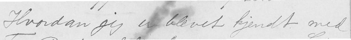Hvordan jeg er blevet kjendt med
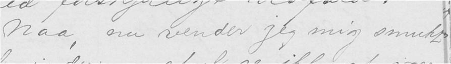Naa, nu vender jeg mig smukt
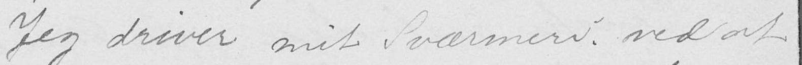Jeg driver mit Sværmeri ved at
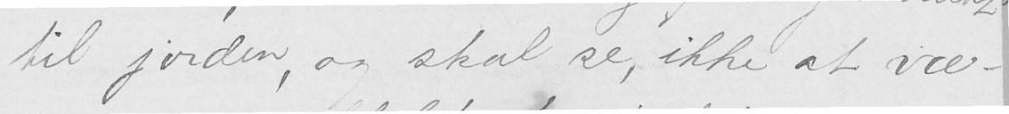til jorden og skal se, ikke at væ-
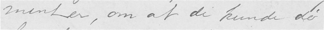menter, om at de kunde dø
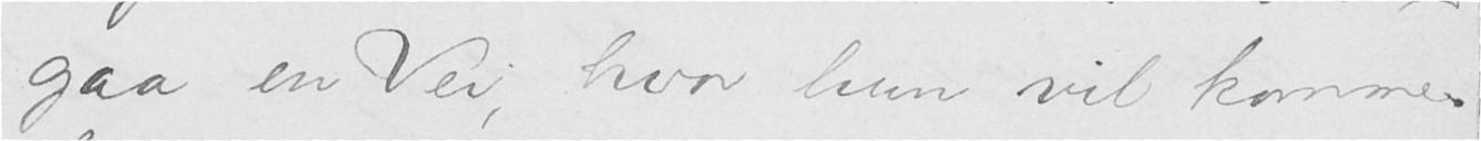gaa en Vei, hvor hun vil komme.
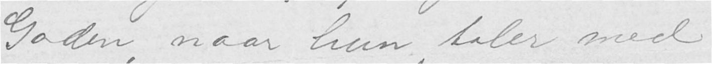Gaden, naar hun taler med
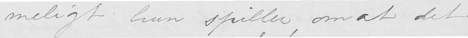meligt hun spiller, om at det
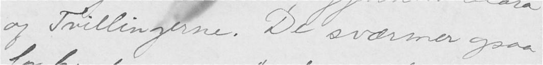og Tvillingerne. De sværmer ogsaa
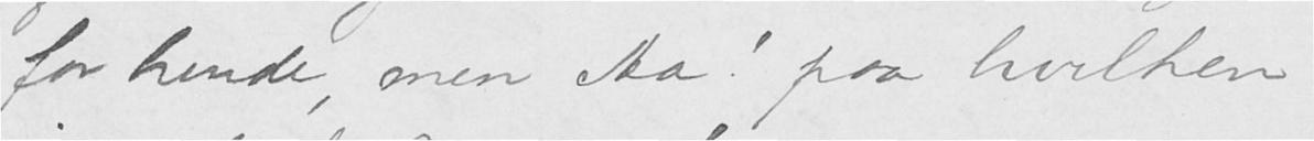for hende, men Aa! paa hvilken
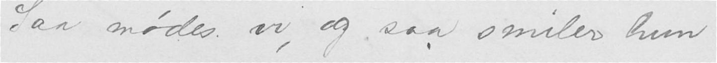Saa mødes vi og saa smiler hun
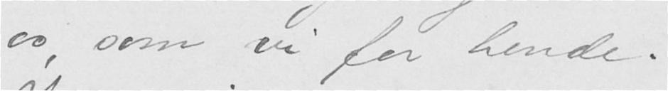os, som vi for hende.
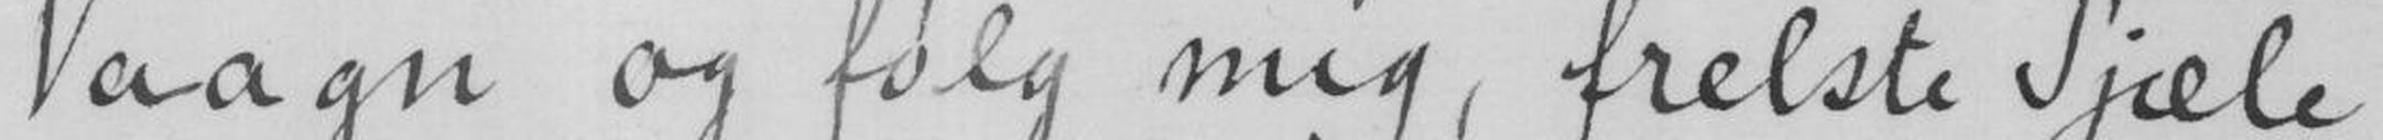Vaagn og følg mig, frelste Sjæle
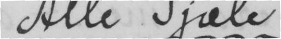Alle Sjæle
